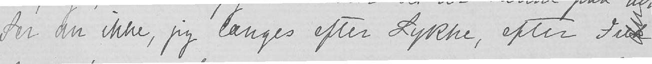Ser du ikke, jeg længes efter Lykke, efter Fred
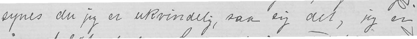synes du jeg er ukvindelig, saa sig det, jeg er
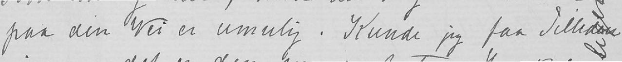paa den Vei er umulig. Kunde jeg faa Tilliden
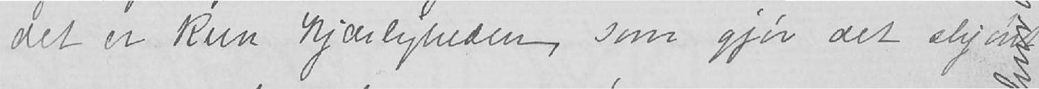det er kun Kjærligheden, som gjør det skjønt,
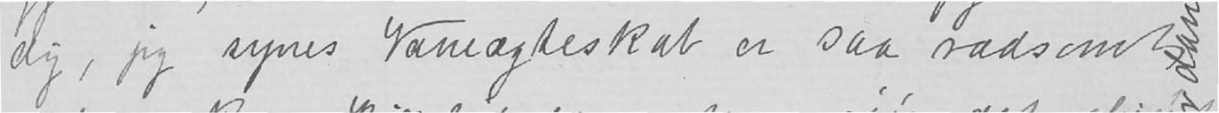dig, jeg synes Vaneægteskabet er saa rædsomt,
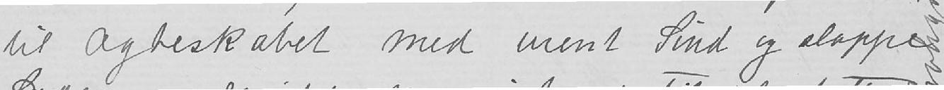til Ægteskabet med urent Sind og slappe
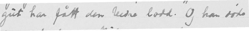gut har fått den bedre lodd. Og han døde
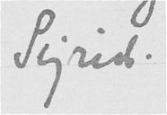Sigrid.
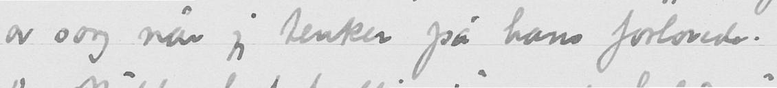av sorg når jeg tenker pa hans forlovede.
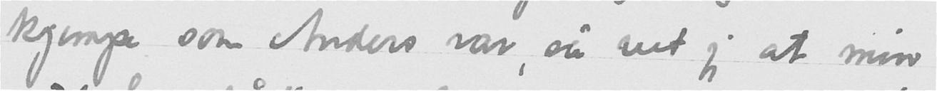kjempe som Anders var, så vet jeg at min
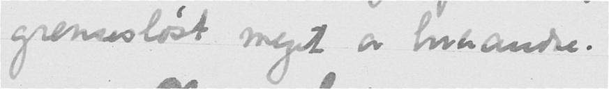grensesløst meget av hverandre.
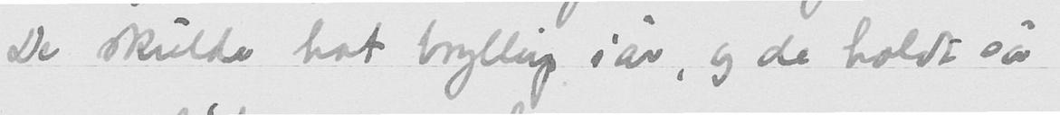De skulde hat bryllup i år, og de holdt så
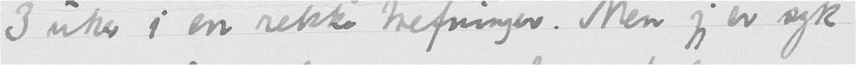3 uker i en rekke trefninger. Men jeg er syk
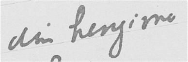din hengivne
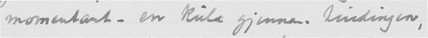momentant - en kule gjennem tindingen,
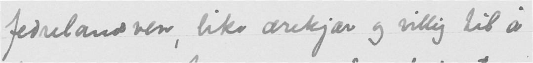fedrelandsven, like ærekjær og villig til å
